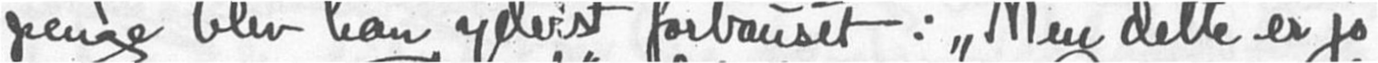penge blev han yderst forbauset :"Men dette er jo
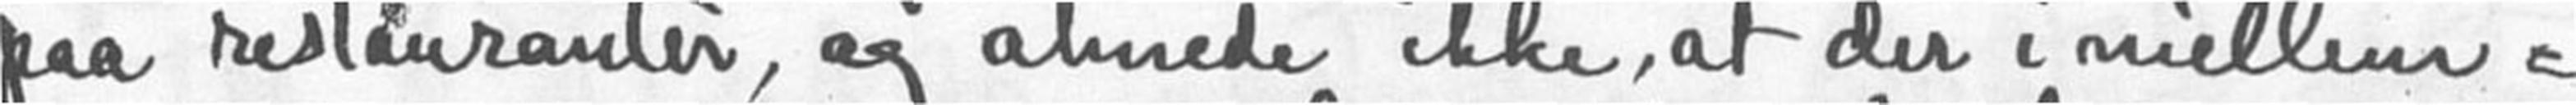paa restauranter, og ahnede ikke, at der imellem
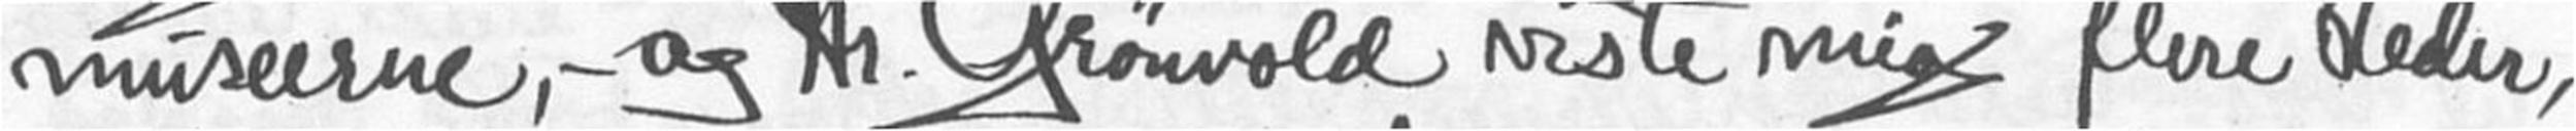museerne, - og Hr. Grønvold viste mig flere steder
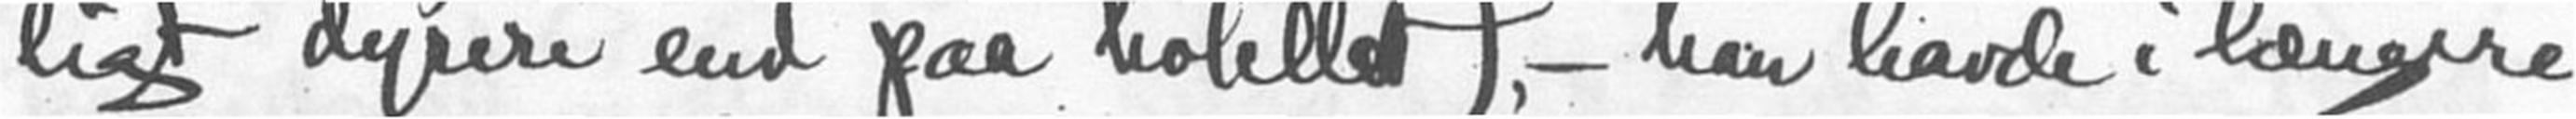ligt dyrere end paa hotellet),- han havde i længere
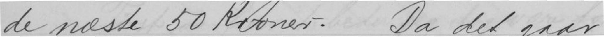de næste 50 Kroner. Da det gaar
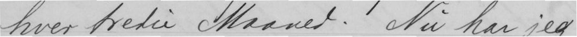hver tredie Maaned. Nu har jeg
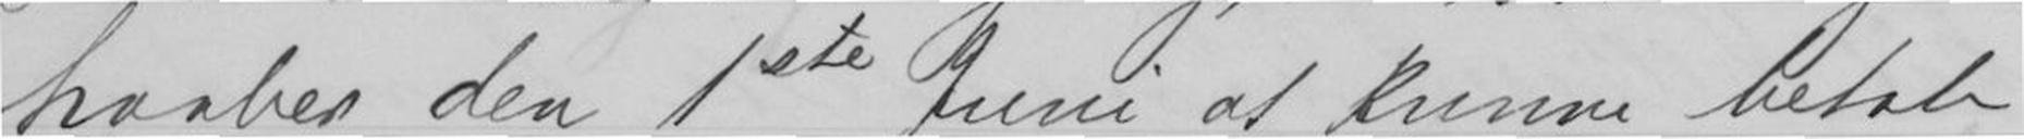haaber den 1ste Juni at kunne betale
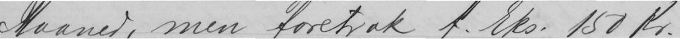Maaned, men foretrak f. Eks. 150 Kr.
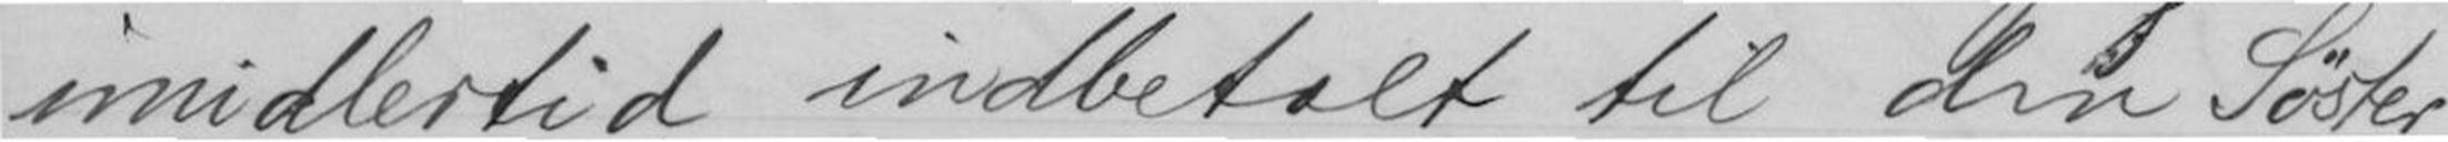imidlertid indbetalt til din Søster,
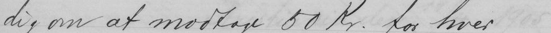dig om at modtage 50 Kr. for hver
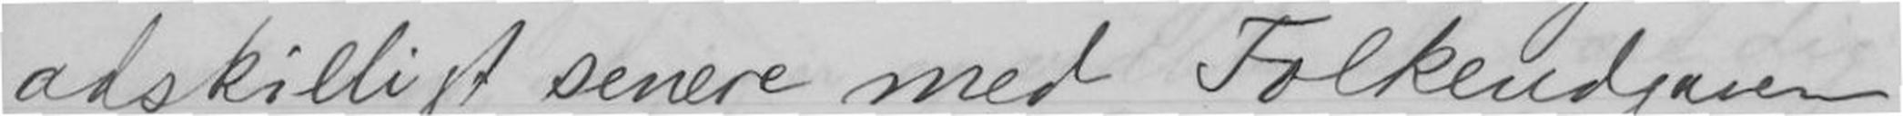adskilligt senere med Folkeudgaven
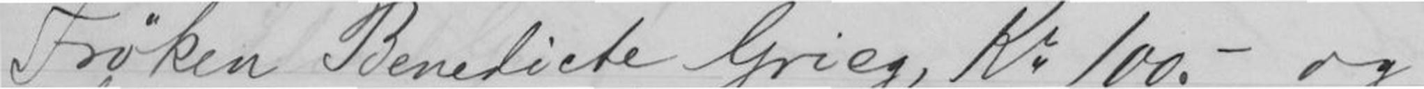Frøken Benedicte Grieg, Kr 100.- og
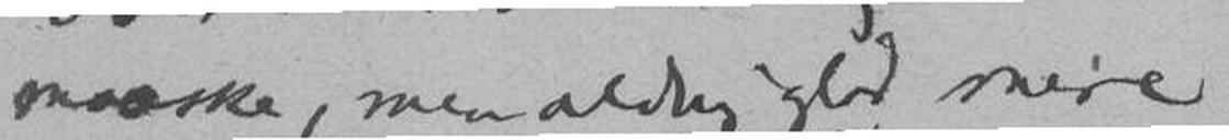maaske, men aldrig glad mere
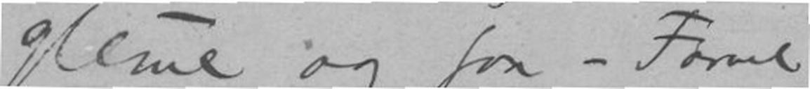gleme og faa - Farvel
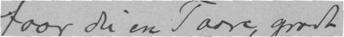faar du en Taare, gjordt
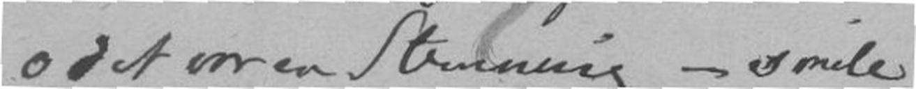O det var en Stemning - smule
